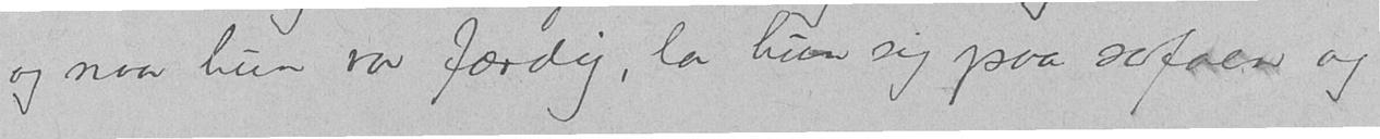og naar hun var færdig, la hun sig paa sofaen og
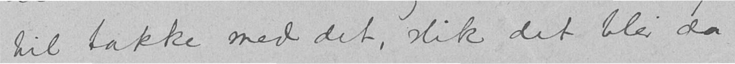til takke med det, slik det blev da
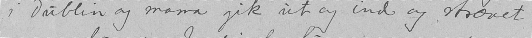i Dublin og mama gik ut og ind og strævet
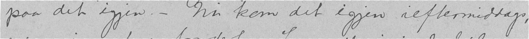paa det igjen. - Nu kom det igjen ieftermiddags,
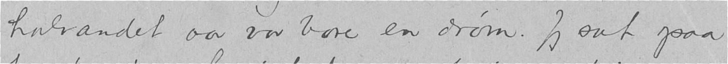halvandet aar var bare en drøm. Jeg sat paa
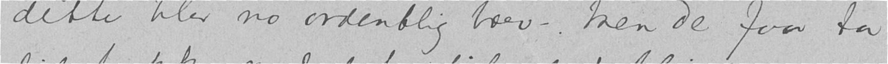dette blir no ordentlig brev –. Men De faar ta
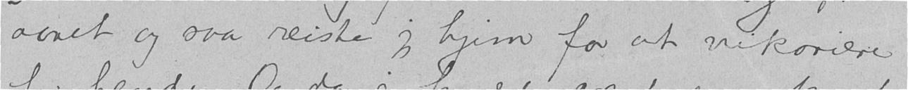aaret og saa reiste jeg hjem for at vikariere
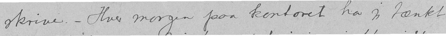skrive. - Hver morgen paa kontoret har jeg tænkt
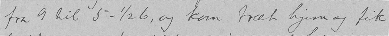fra 9 til 5 - ½ 6, og kom træt hjem og fik
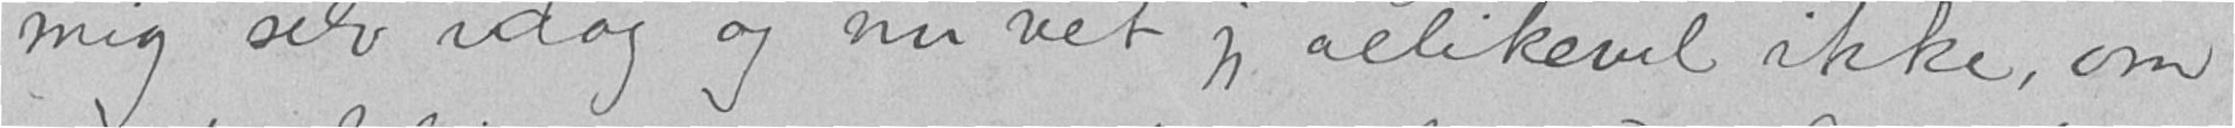mig selv idag og nu vet jeg allikevel ikke, om
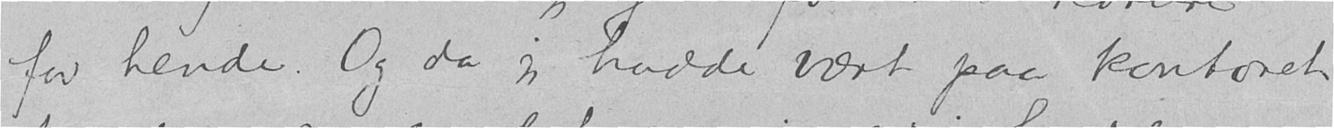for hende. Og da jeg hadde vært paa kontoret
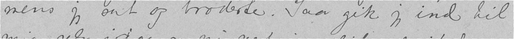mens jeg sat og broderte. Saa gik jeg ind til
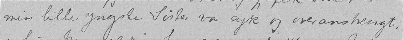min lille yngste Søster var syk og overanstrengt,
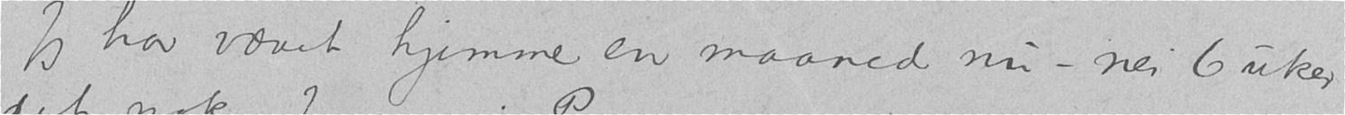Jeg har været hjemme en maaned nu – nei 6 uker
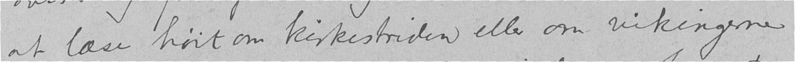at læse høit om kirkestriden eller om vikingerne
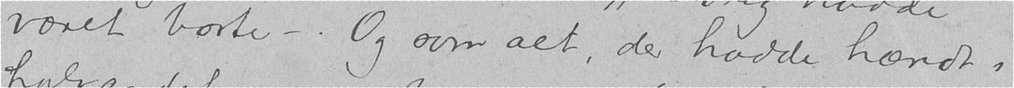været borte -. Og som alt, der hadde hændt i
